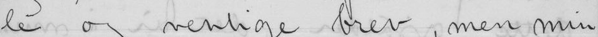le og venlige brev, men min
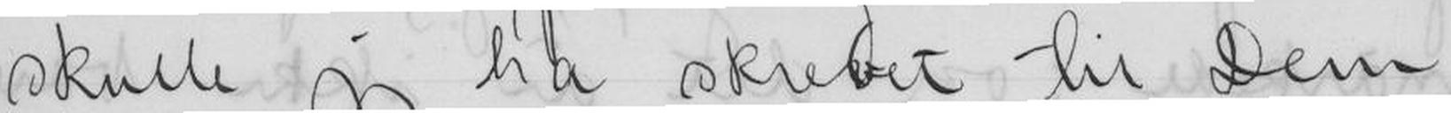skulle jeg ha skrevet til Dem
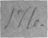176.
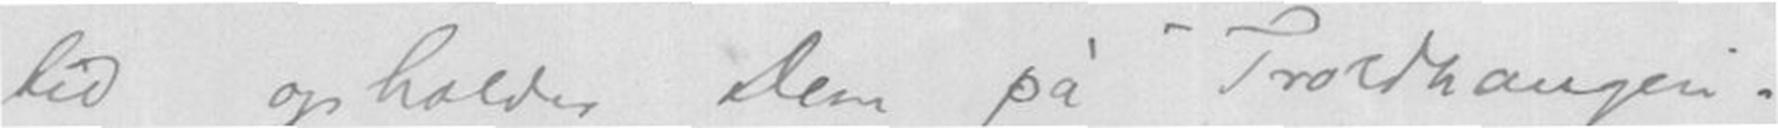tid opholder Dem på "Troldhaugen".
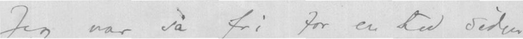Jeg var så fri for en tid siden
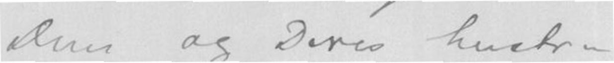Dem og Deres hustru
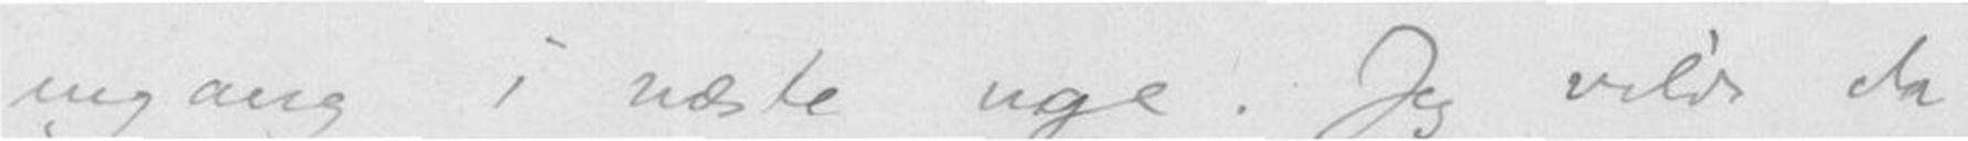engang i næste uge. Jeg vilde da
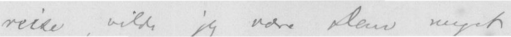reise, vilde jeg være Dem meget
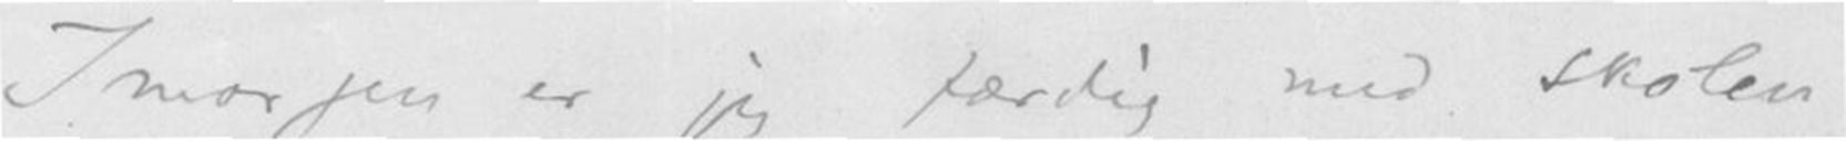Imorgen er jeg færdig med skolen,
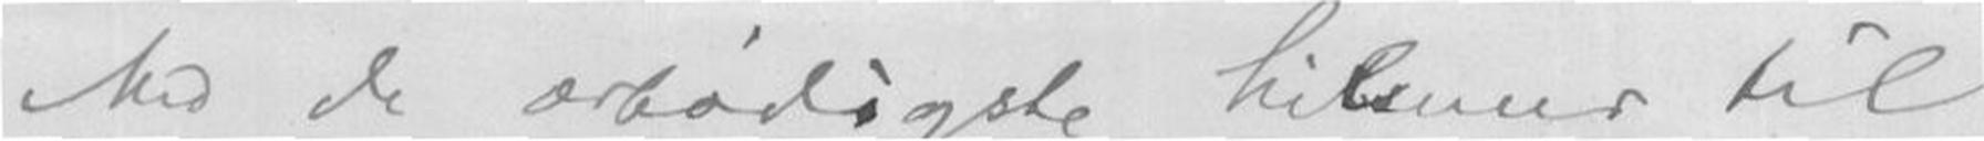Med de ærbødigste hilsener til
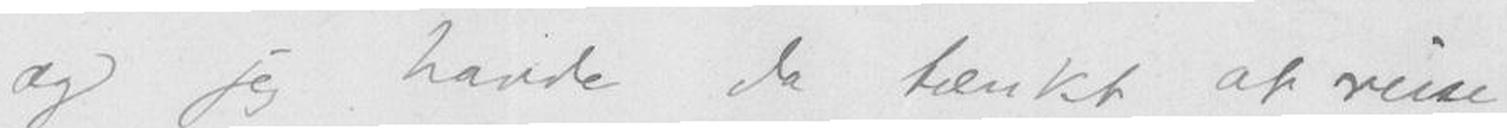og jeg havde da tænkt at reise
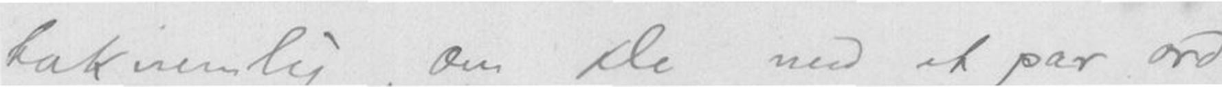taknemlig, om De med et par ord
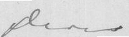Deres
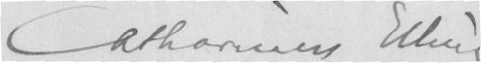Catharinus Elling
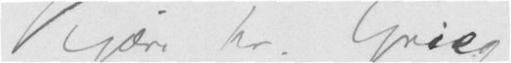Kjære hr. Grieg!
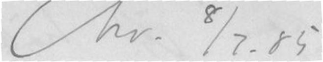Chr. 8/7.85
